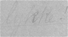lykke!
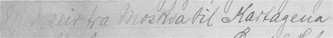Med seir fra Moskva til Kartagena
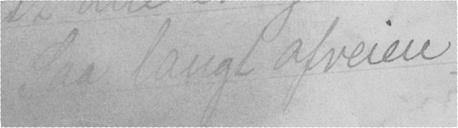Saa langt afveien
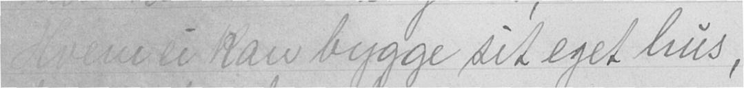Hvem ei kan bygge sit eget hus,
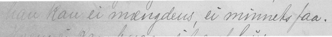han kan ei mængdens, ei minnets faa.
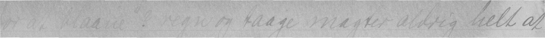for at blaane"? regn og taage magter aldrig helt at
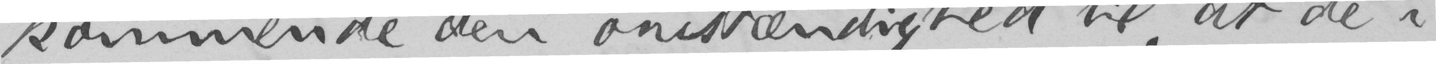kommende den omstændighed til, at de i
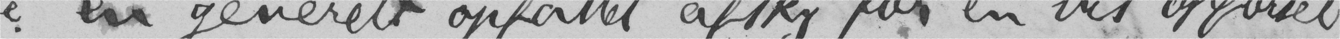en generelt opfattet afsky for en vis opførsel
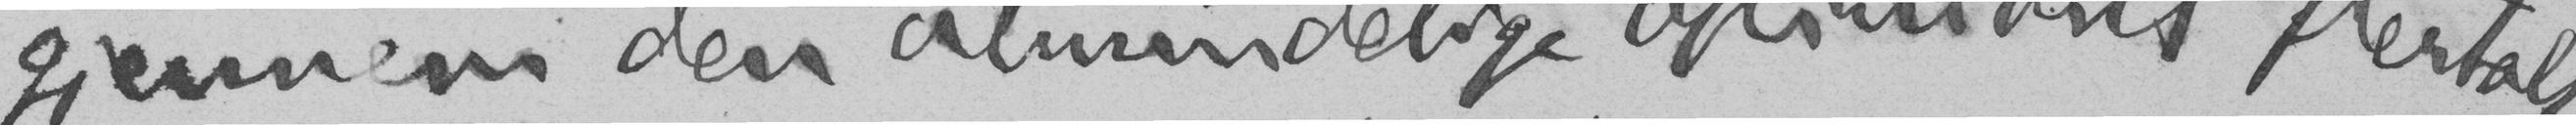gjennem den almindelige opinions flertals-
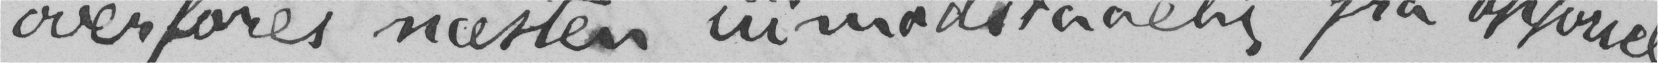overføres næsten uimodstaaelig fra opførselen
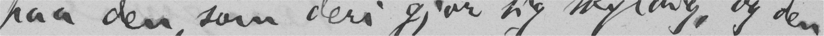paa den, som deri gjør sig skyldig, og den
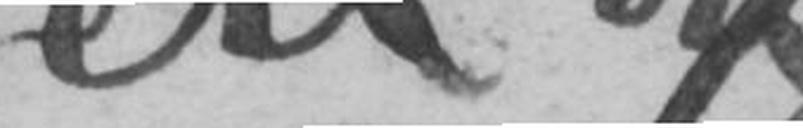en af
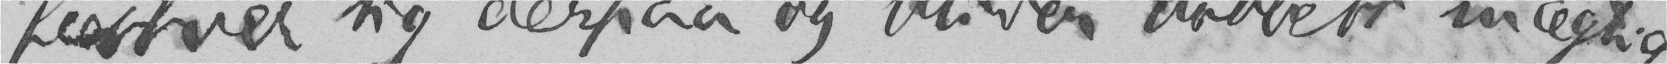fæstner sig derpaa og bliver dobbelt mægtig
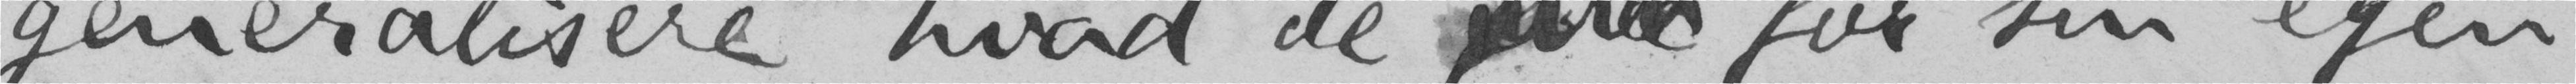generalisere, hvad de <uleseleg med blekkflekk over> for sin egen
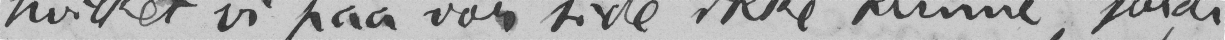hvilket vi paa vor side ikke kunne, fordi
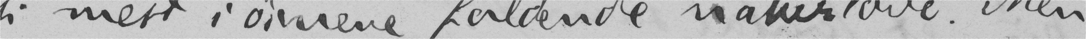mest i øinene faldende naturlove. Men
